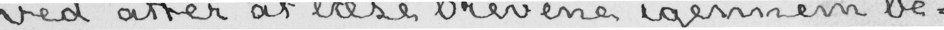ved atter at læse brevene igennem be-
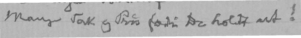Mange Tak og Pris fordi De holdt ut!
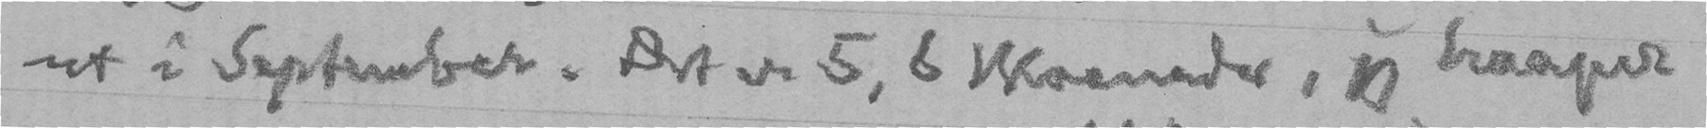ut i September. Det er 5, 6 Maaneder, jeg haaper
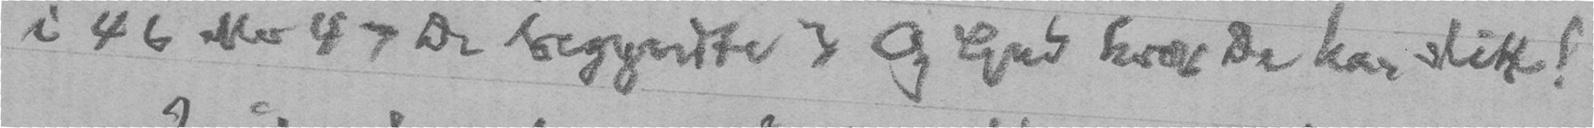i 46 eller 47 De begyndte? Og Gud hvor De har slitt!
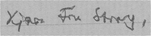Kjære Fru Stray,
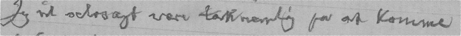Jeg vil selvsagt være taknemlig for at komme
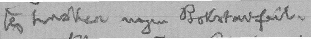jeg husker nogen Bokstavfeil.
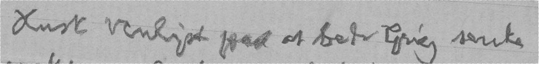Husk venligst paa at bede Grieg sende
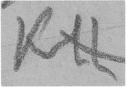KH
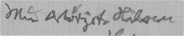Min ærbødigste Hilsen
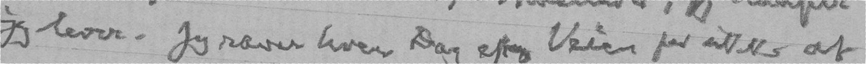jeg lever. Jeg raver hver Dag efter Veien for ikke at
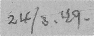24/3. 49.
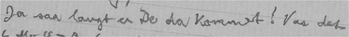Ja saa langt er De da kommet! Var det
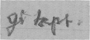gi tapt.
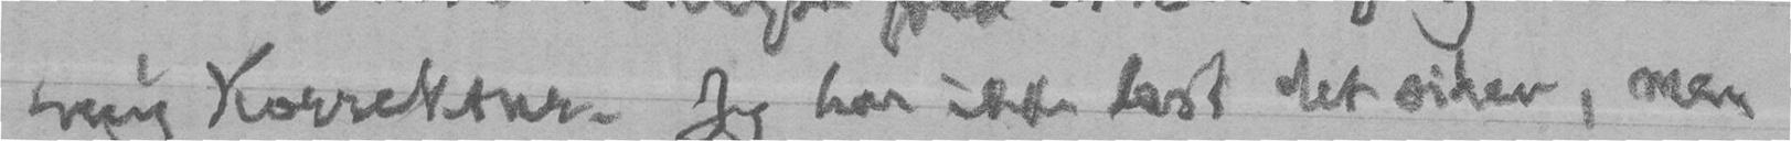mig Korrektur. Jeg har ikke læst det siden, men
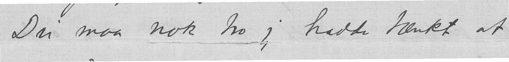Du maa nok tro jeg hadde tænkt at
